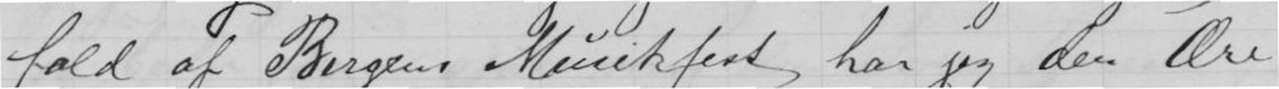fald af Bergens Musikfest har jeg den Ære
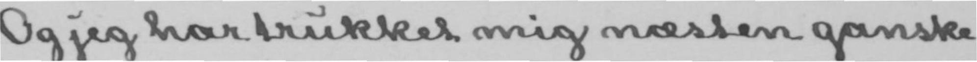Og jeg har trukket mig næsten ganske
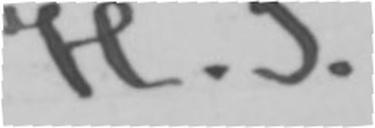H. I.
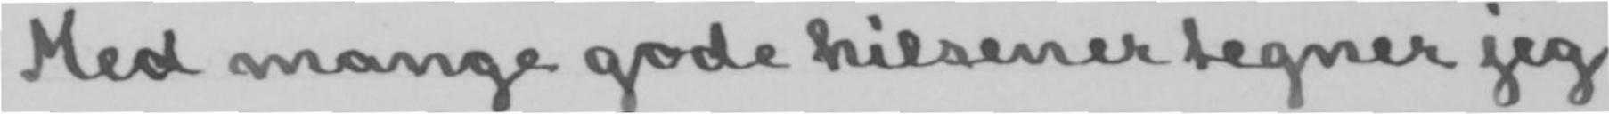Med mange gode hilsener tegner jeg
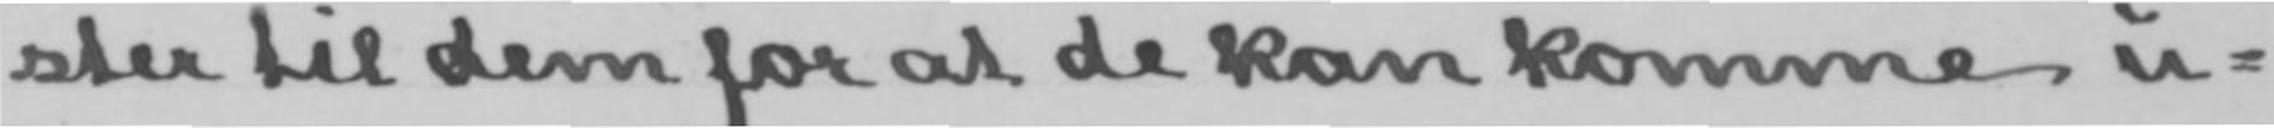ster til dem for at de kan komme u-
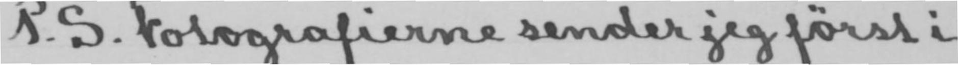P. S. Fotografierne sender jeg først i
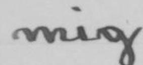mig
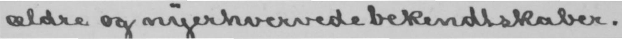ældre og nyerhvervede bekendtskaber.
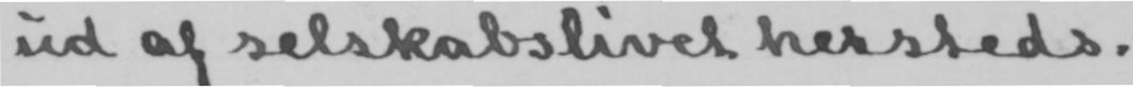ud af selskabslivet hersteds.
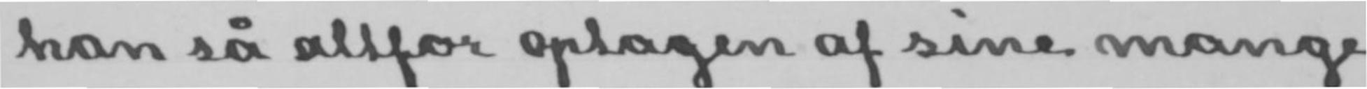han så altfor optagen af sine mange
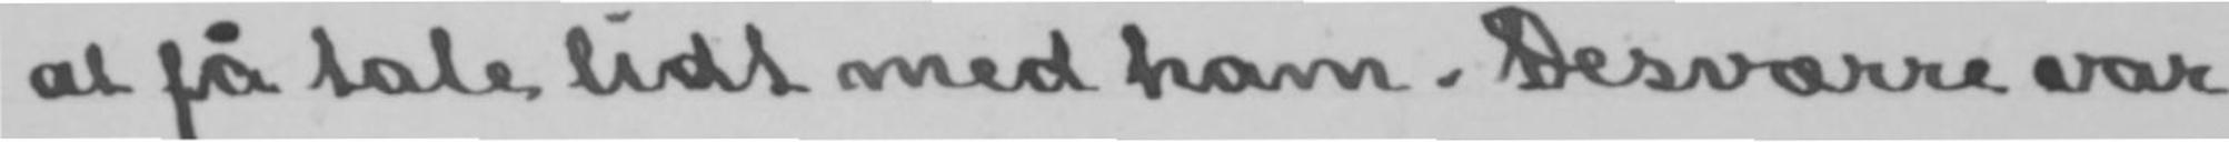at få tale lidt med ham. Desværre var
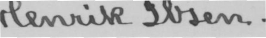Henrik Ibsen.
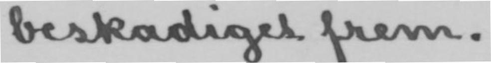beskadiget frem.
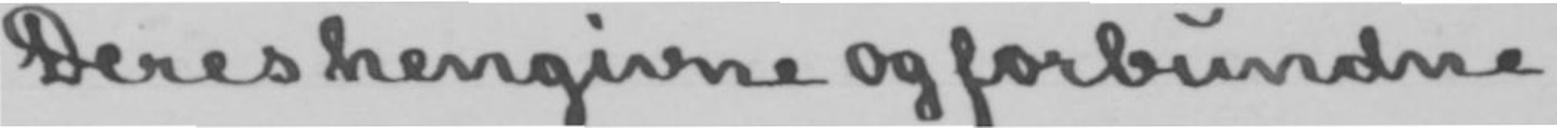Deres hengivne og forbundne
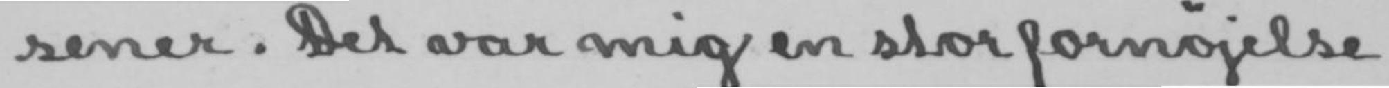sener. Det var mig en stor fornøjelse
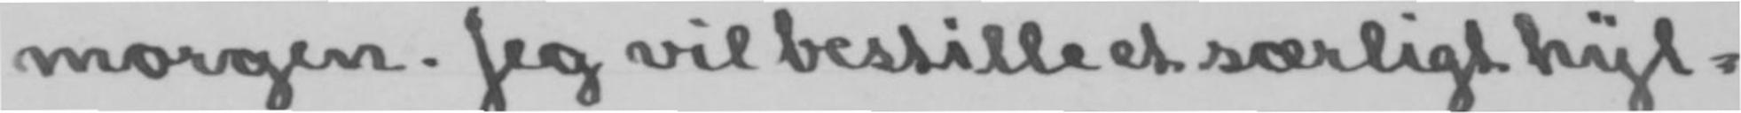morgen. Jeg vil bestille et særligt hyl-
